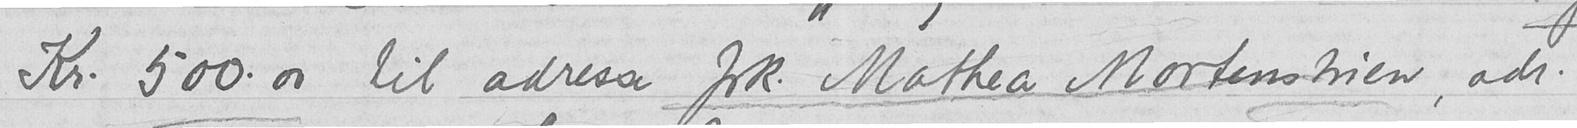Kr. 500.00 til adresse frk Mathea Mortenstuen, adr.
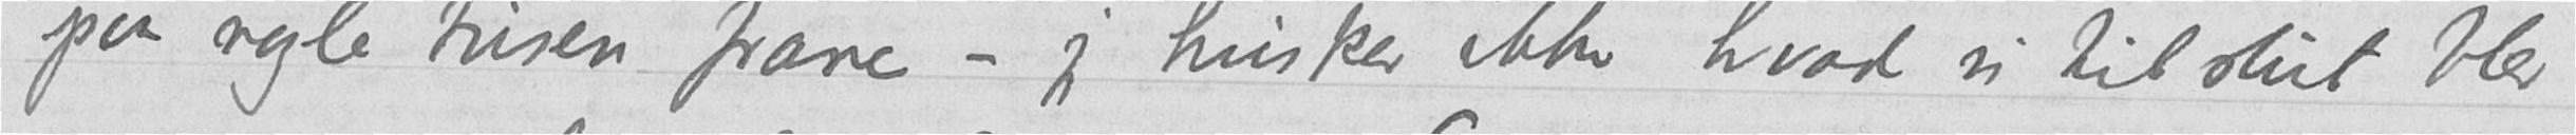paa nogle tusen franc – jeg husker ikke hvad vi til slut blev
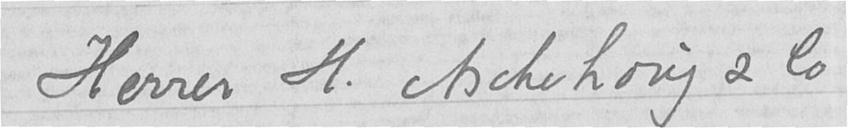Herrer H. Aschehoug & Co,
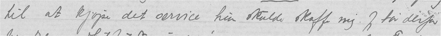til at kjøpe det service hun skulde skaffe mig. Jeg tør derfor
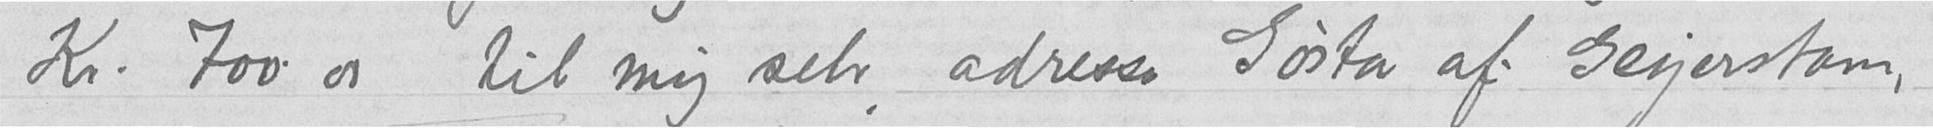Kr. 700.00 til mig selv, adresse Gøsta af Geijerstam,
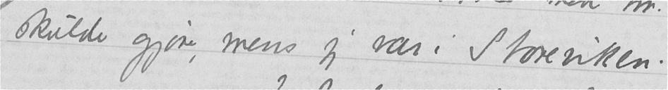skulde gjøre, mens jeg var i Storeviken.
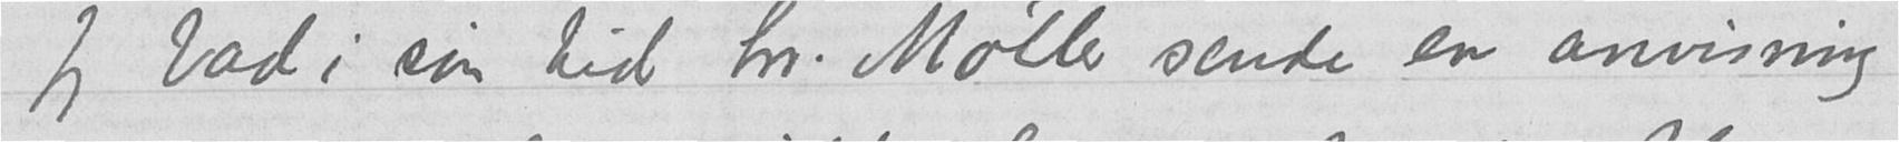Jeg bad i sin tid hr. Møller sende en anvisning
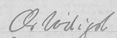Ærblødigst
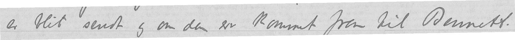er blit sendt og om den er kommet frem til Bennett.
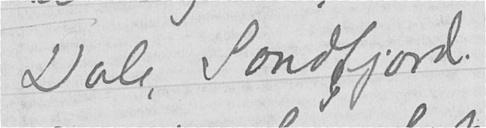Dale, Søndfjord.
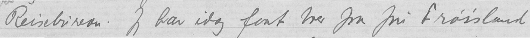Reisebureau. Jeg har idag faat brev fra fru Frøisland
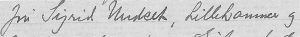fru Sigrid Undset, Lillehammer og
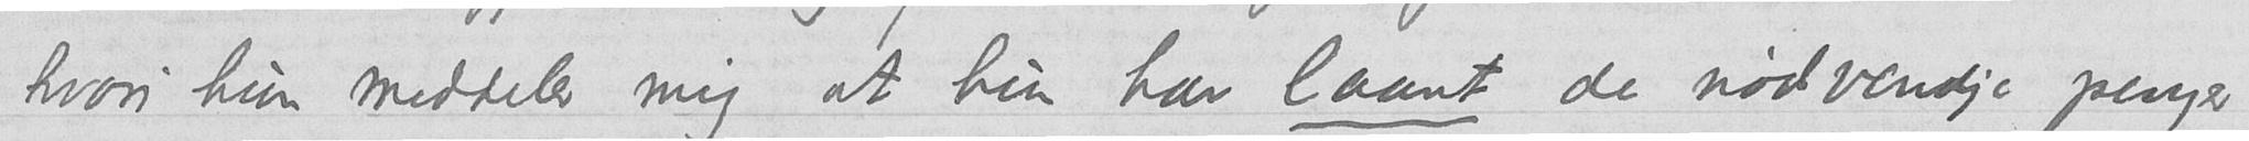hvori hun meddeler mig at hun har laant de nødvendige penger
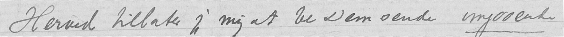Herved tillater jeg mig at be Dem sende omgaaende
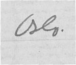Oslo.
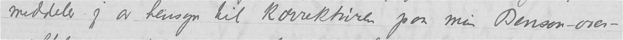meddeler jeg av hensyn til korrekturen paa min Benson-over-
